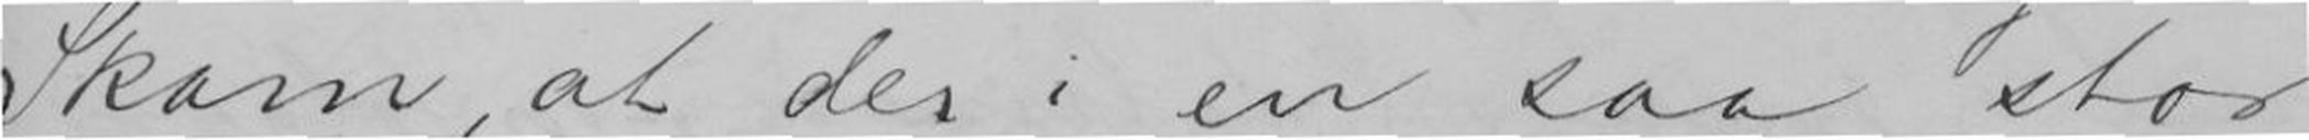Skam, at der i en saa stor
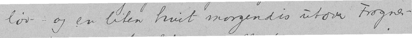løv- og en liten hvit morgendis utover Frogner-
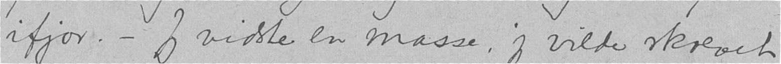ifjor. - Jeg vidste en masse, jeg vilde skrevet
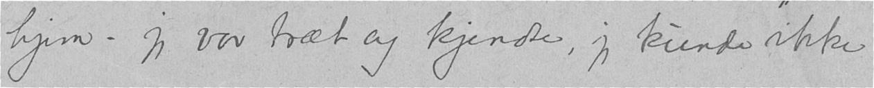hjem - jeg var træt og kjendte, jeg kunde ikke
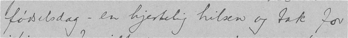fødselsdag - en hjertelig hilsen og tak for
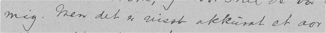mig. Men det er visst akkurat et aar
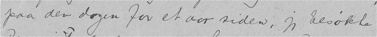paa den dagen for et aar siden, jeg besøkte
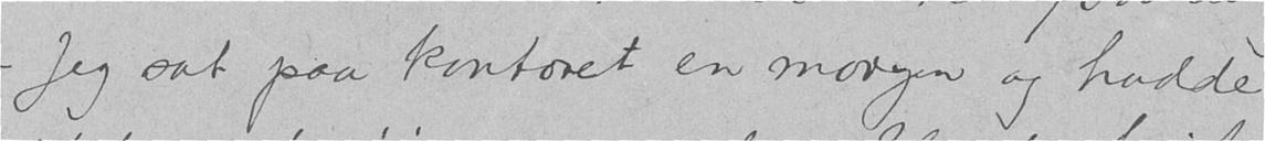- Jeg sat paa kontoret en morgen og hadde
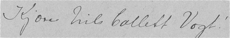Kjære Nils Collett Vogt!
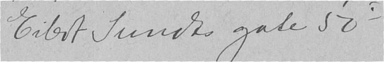Eilert Sundts gate 50l
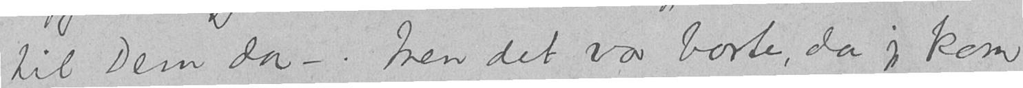til Dem da -. Men det var borte, da jeg kom
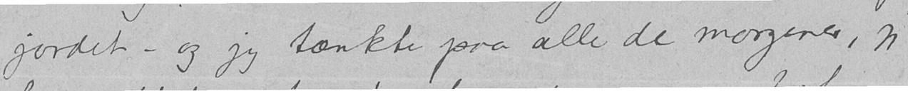jordet - og jeg tænkte paa alle de morgener, jeg
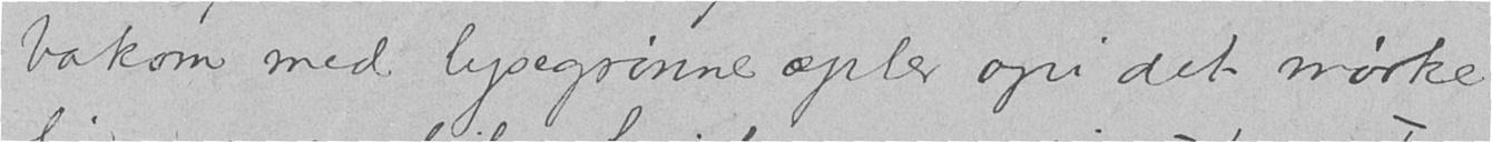bakom med lysegrønne epler opi det mørke
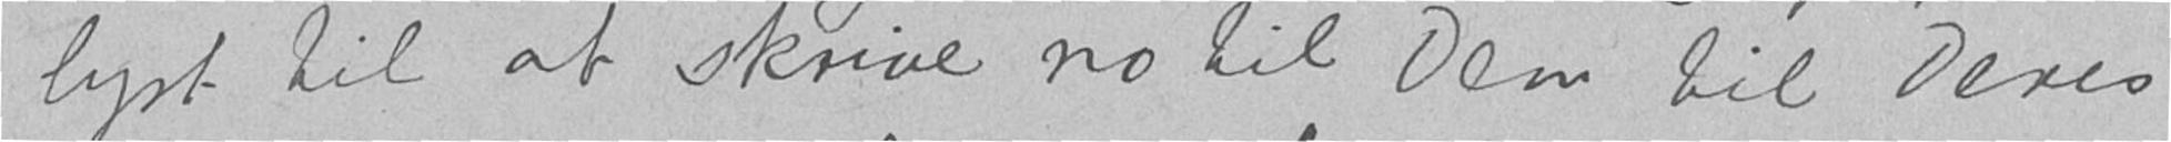lyst til at skrive no til Dem til Deres
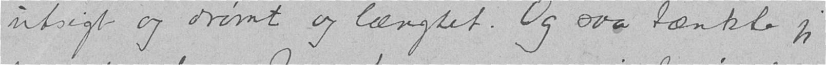utsigt og drømt og længtet. Og saa tænkte jeg
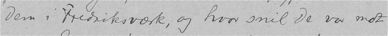Dem i Fredriksværk, og hvor snil De var mot
